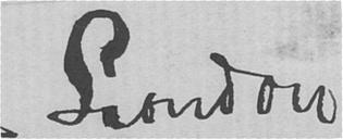London
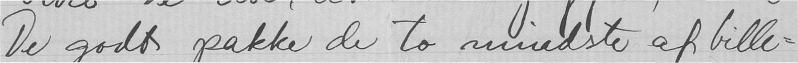De godt pakke de to mindste af bille-
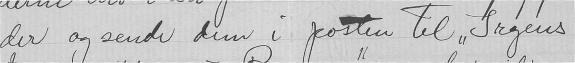der og sende dem i posten til "Irgens"
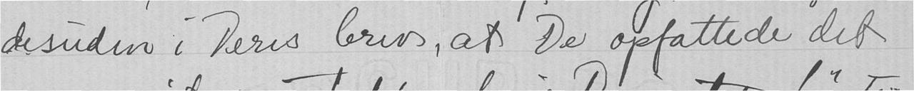desuden i Deres brev, at De opfattede det
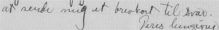at sende mig et brevkort til svar.
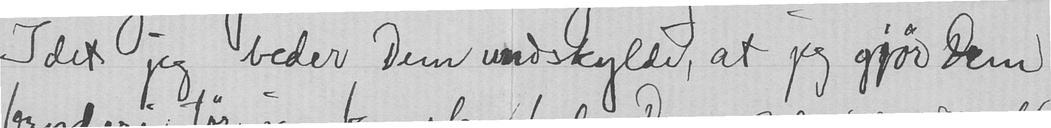I det jeg beder Dem undskylde, at jeg gjør Dem
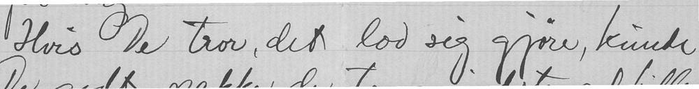Hvis De tror, det lod sig gjøre, kunde
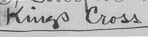Kings Cross
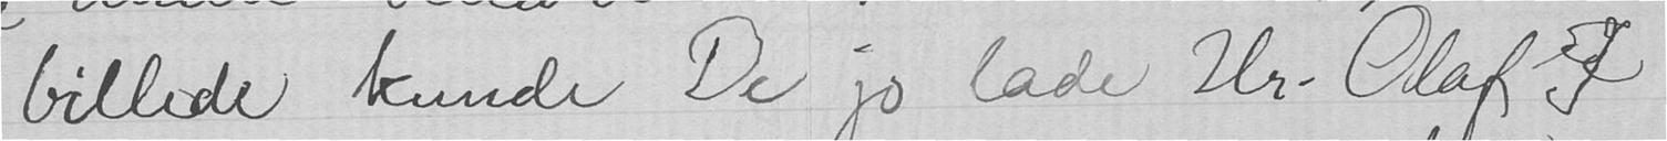billede kunde De jo lade Hr. Olaf J
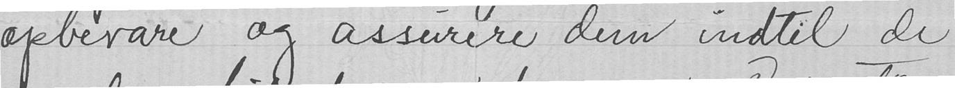opbevare og assurere dem indtil de
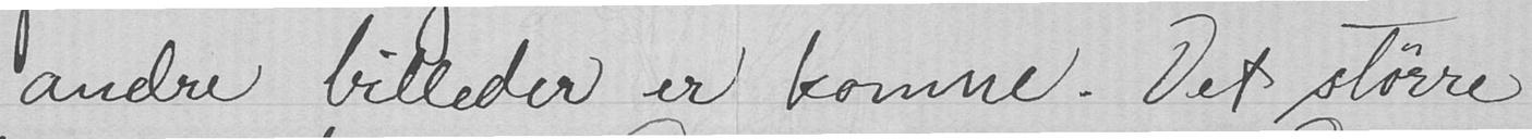andre billeder er komne. Det større
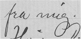fra mig.
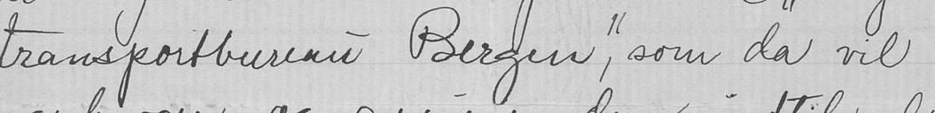transportbureau Bergen", som da vil
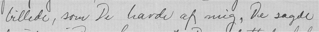billede, som De havde af mig, De sagde
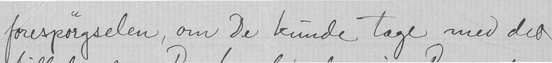forespørgselen, om De kunde tage med det
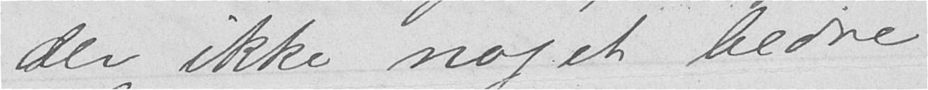der ikke noget bedre
